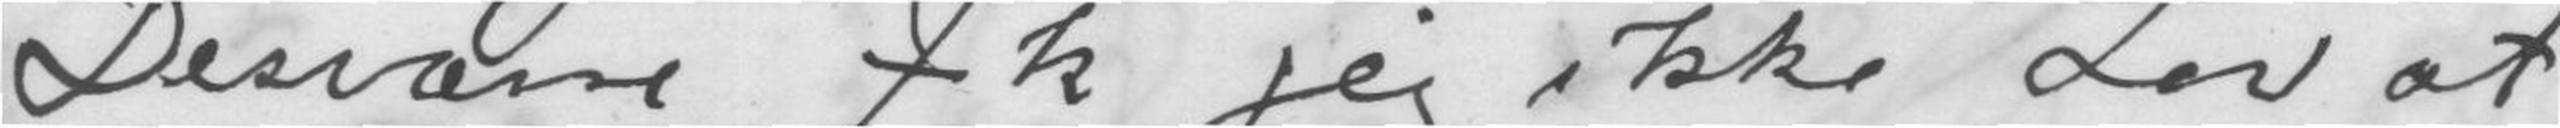Desværre fik jeg ikke Lov at
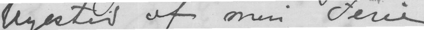Ugestid af min Ferie
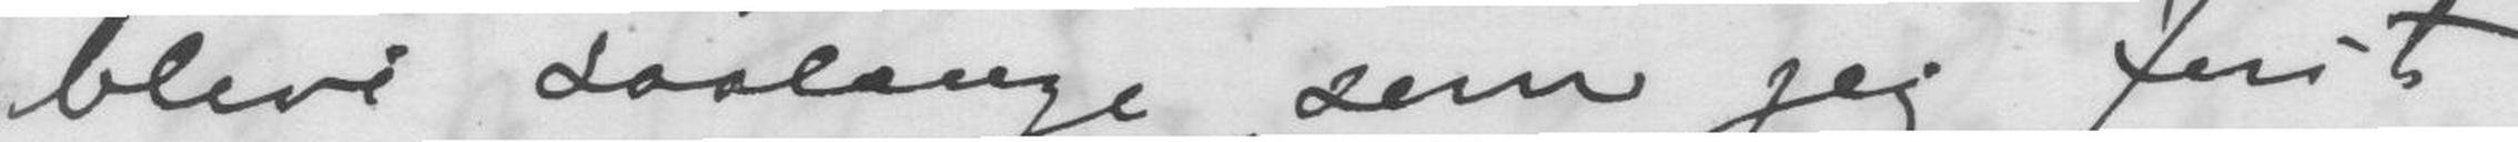blive saalænge, som jeg først
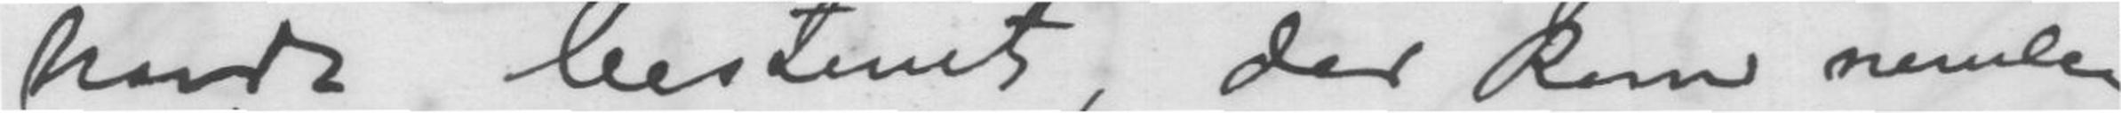havde bestemt, der kom nemlig
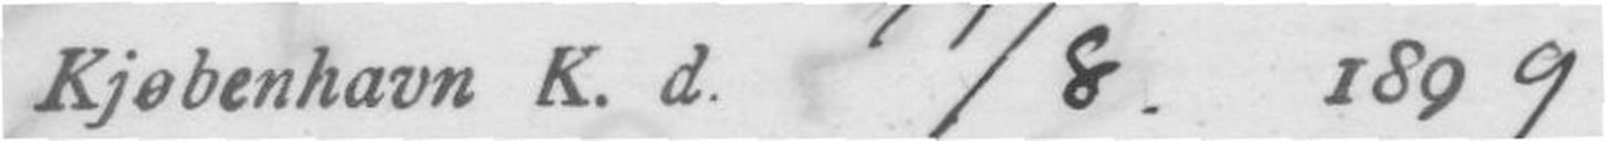København K.d. 19/8. 1899.
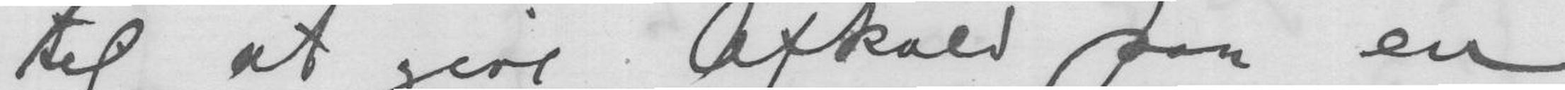til at gøre Afkald paa en
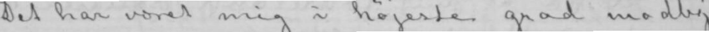Det har været mig i højeste grad modby-
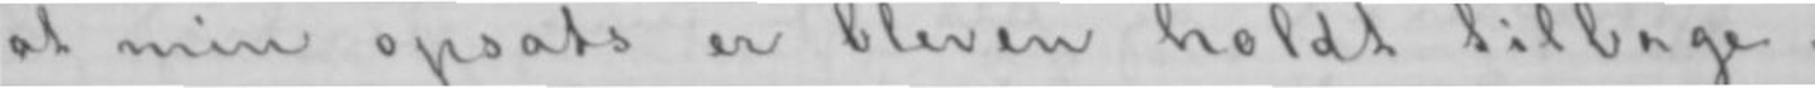at min opsats er bleven holdt tilbage.
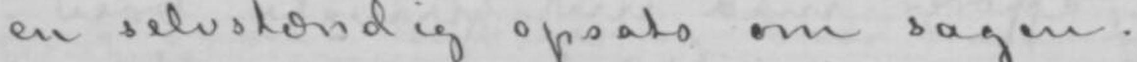en selvstændig opsats om sagen.
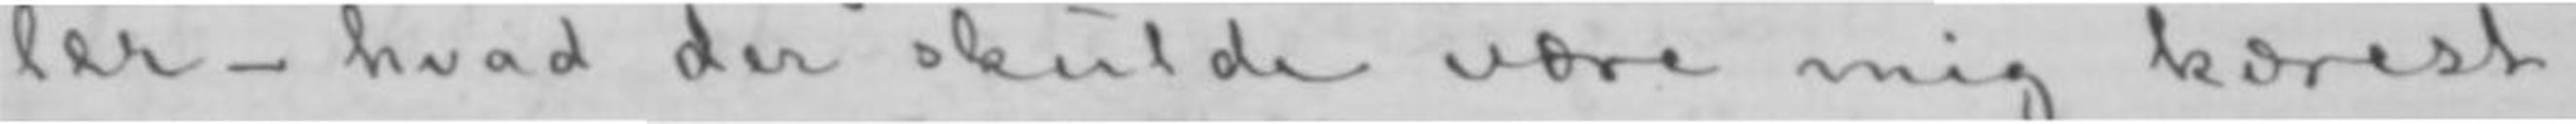ler- hvad der skulde være mig kærest-
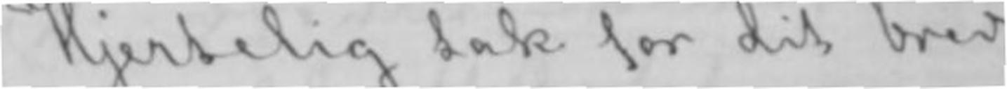Hjertelig tak for dit brev,
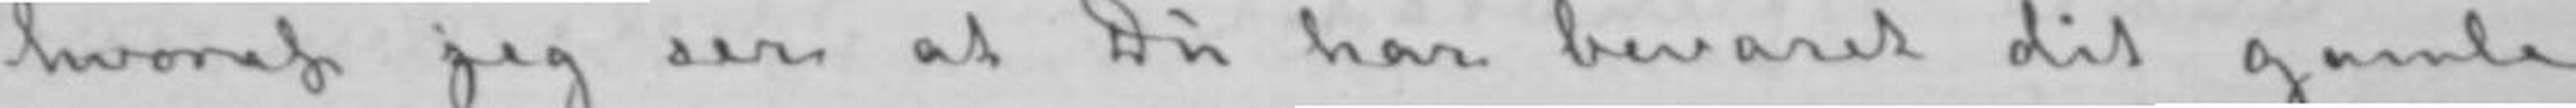hvoraf jeg ser at Du har bevaret dit gamle
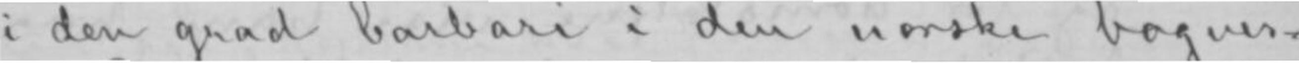i den grad barbari i den norske bogver-
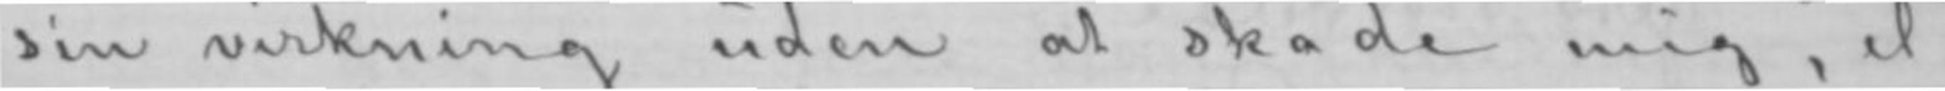sin virkning uden at skade mig, el-
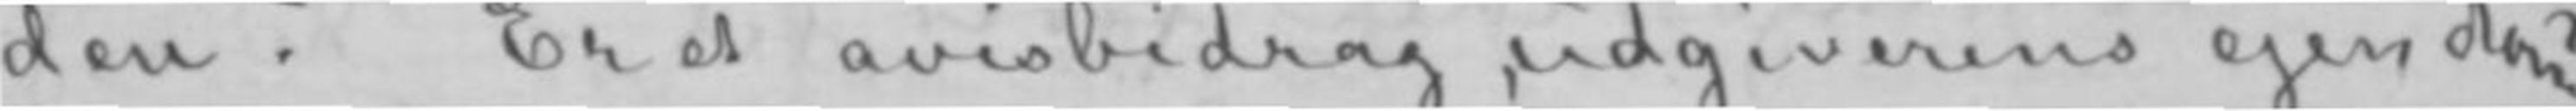den? Er et avisbidrag udgiverens ejendom?
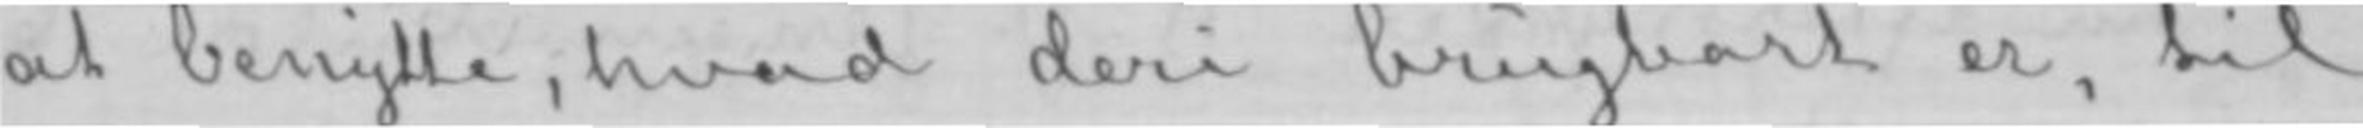at benytte, hvad deri brugbart er, til
